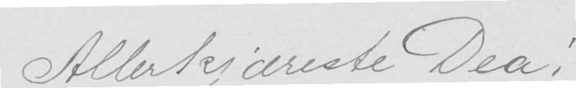Allerkjæreste Dea!
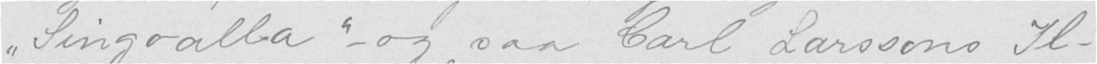"Singoalla" og saa Carl Larssons Il-
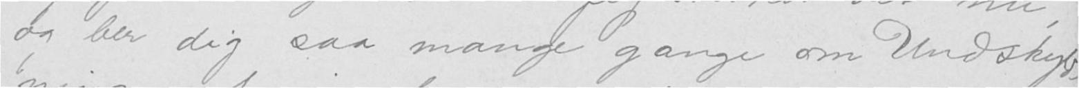og ber dig saa mange ganger om Undskyld-
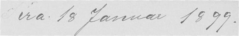Chra. 18 Januar 1899.
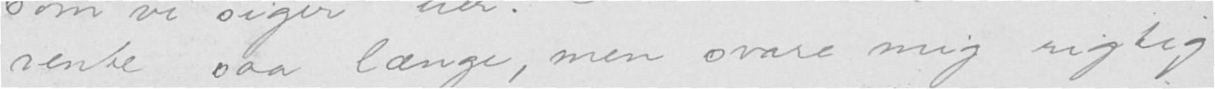vente saa længe, men svare mig rigtig
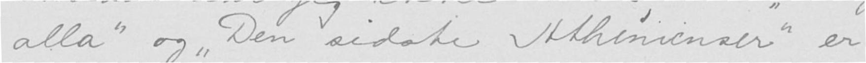alla" og "Den sidste Athenienser" er
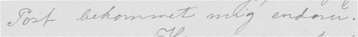Post bekommet mig endnu.
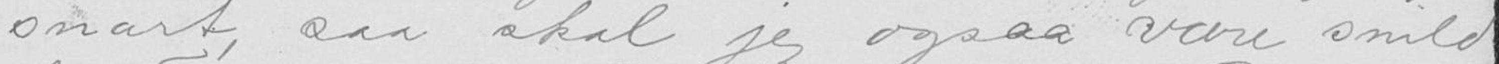snart, saa skal jeg ogsaa være snild
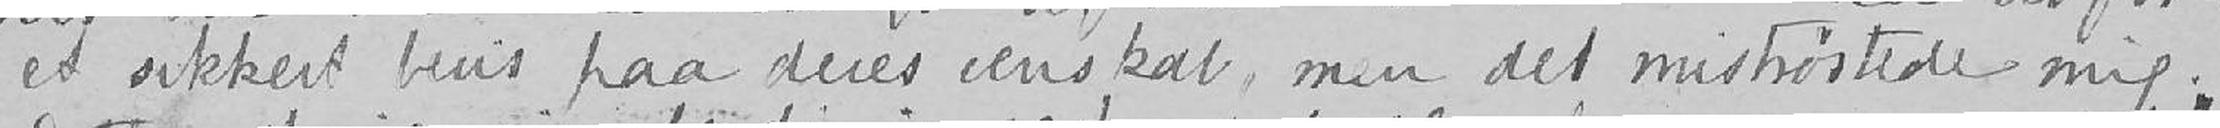et sikkert bevis paa deres venskab, men det mistrøstede mig.
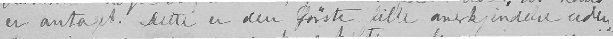er antaget. Dette er den første lille anerkjendelse uden-
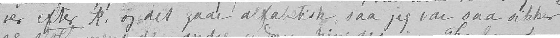ver efter K. og det gaar alfabetisk, saa jeg var saa sikker
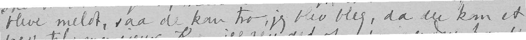bleve meldt, saa de kan tro, jeg blev bleg, da der kom et
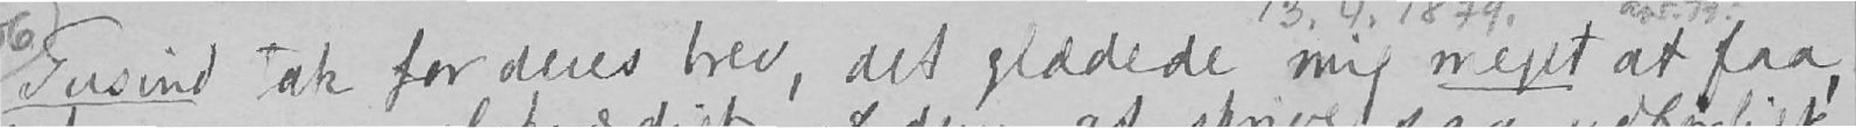Tusind tak for deres brev, det glædede mig meget at faa,
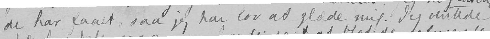de har faaet, saa jeg har lov at glæde mig. Jeg ventede
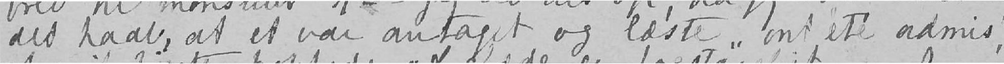det haab, at et var antaget og læste «ont été admis»,
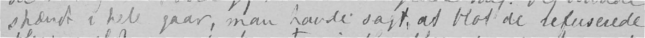spændt i hele gaar, man havde sagt, at blot de refuserede
